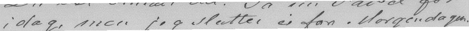i dag, men jeg slutter ei for Morgendagen
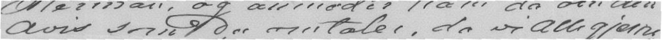Avis som Du omtaler, da vi Alle gjerne
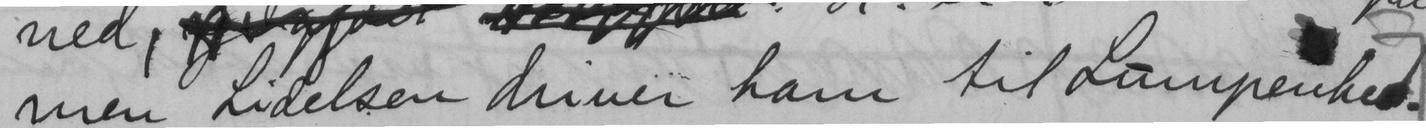men Lidelsen driver ham til Lumpenhed.
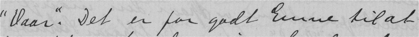"Vaar". Det er for godt Emne til at
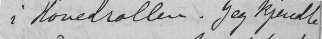i Hovedrollen. Jeg kjendte
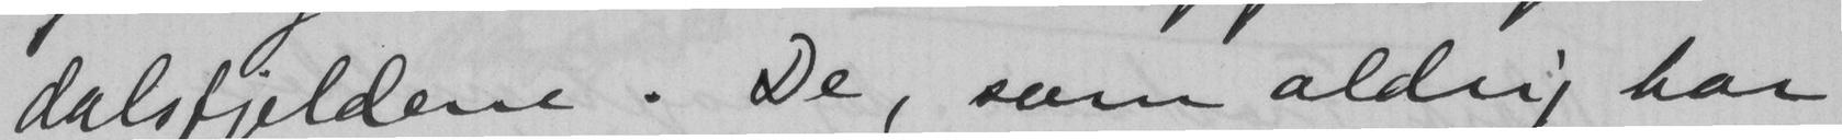dalsfjeldene. De, som aldrig har
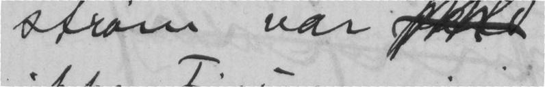strøm var
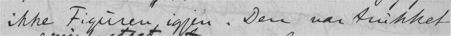ikke Figuren igjen. Den var trukket
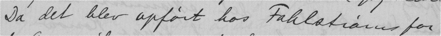Da det blev opført hos Fahlstrøms for
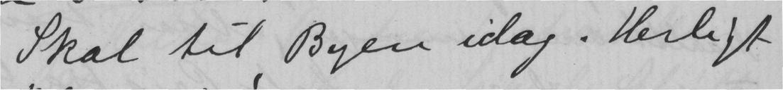Skal til Byen idag. Herligt
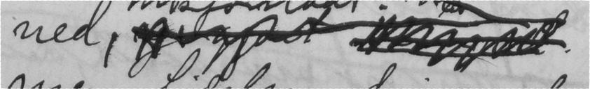ned,
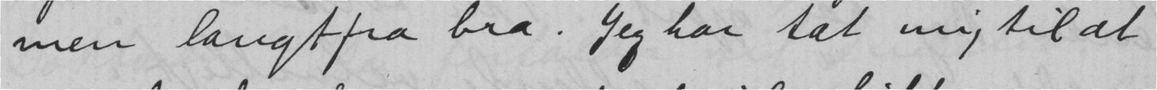men langtfra bra. Jeg har tat mig til at
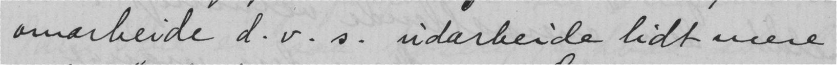omarbeide d. v. s. udarbeide lidt mere
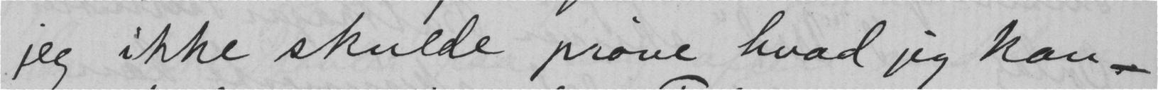jeg ikke skulde prøve hvad jeg kan -
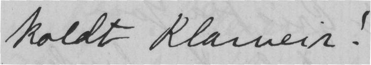koldt klarveir!
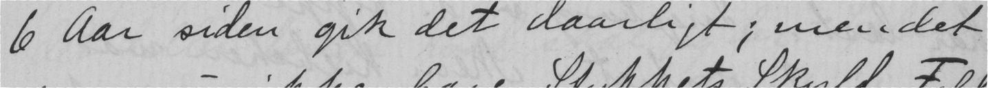6 aar siden gik det daarligt; men det
